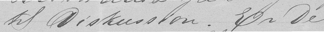til Diskussion. Er De
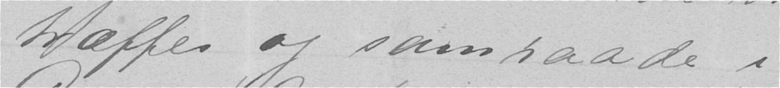tæffes og samraade i
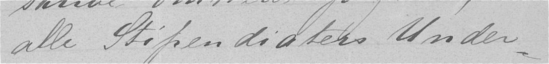alle Stipendiaters Under-
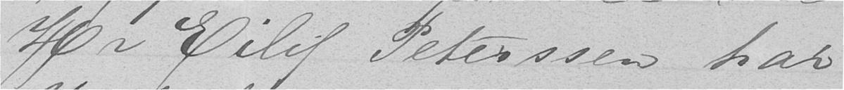Hr Eilif Peterssen har
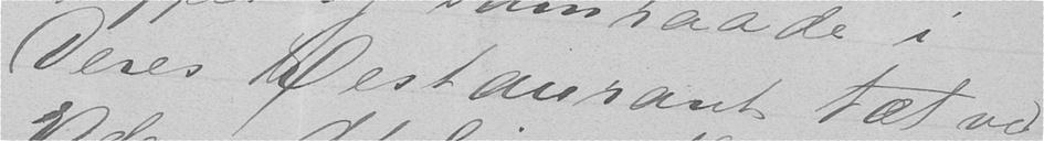Deres Restaurant tæt ved
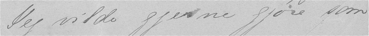Jeg vilde gjerne gjøre som
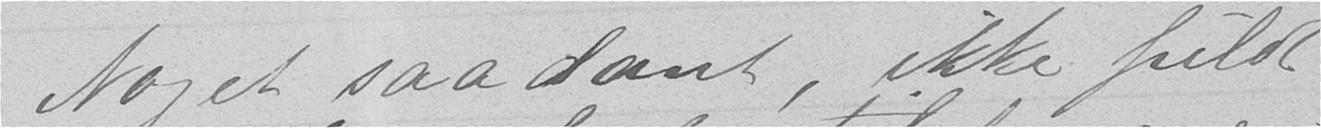Noget saadant, ikke fuldt
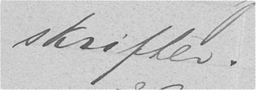skrifter.
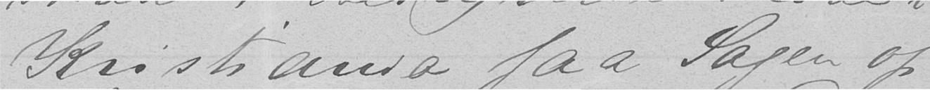Kristiania faa Sagen op
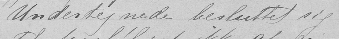Undertegnede besluttet sig
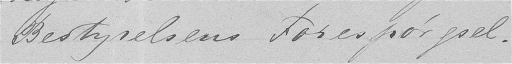Bestyrelsens Forespørgsel.
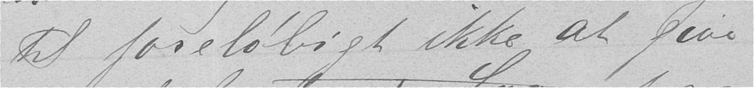til foreløbigt ikke at give
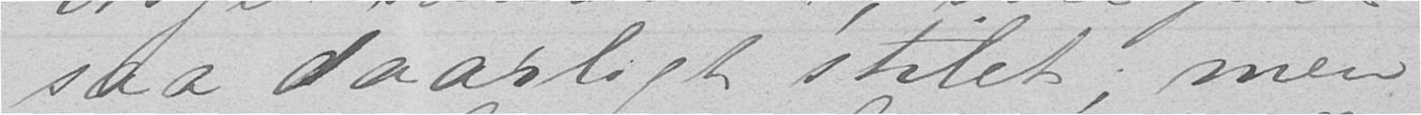saa daarligt stilet; men
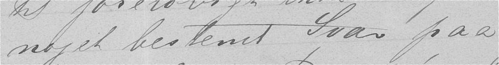noget bestemt Svar paa
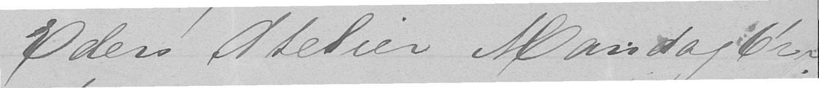Eders Atelier Mandag 6'
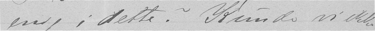enig i dette? Kunde vi ikke
